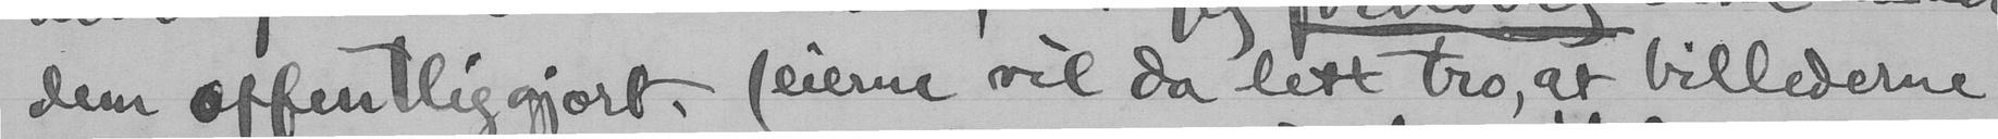dem affentliggjort, (eierne vil da lett tro, at billederne
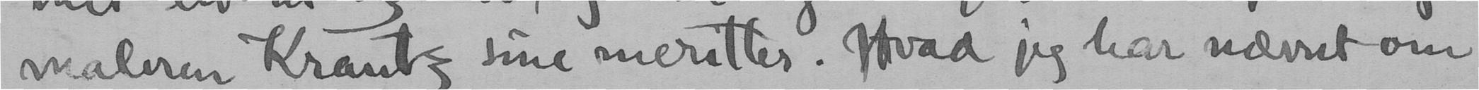maleren Krantz sine meritter. Hvad jeg har nærnt om
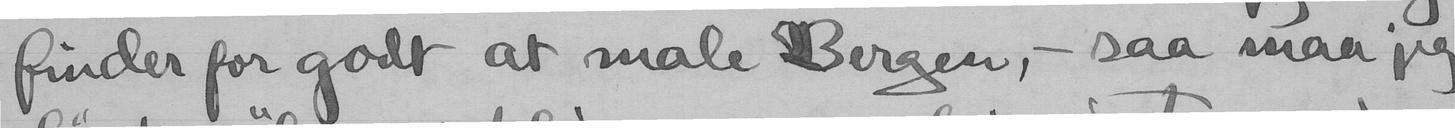finder for godt at male Bergen, - saa maa jeg
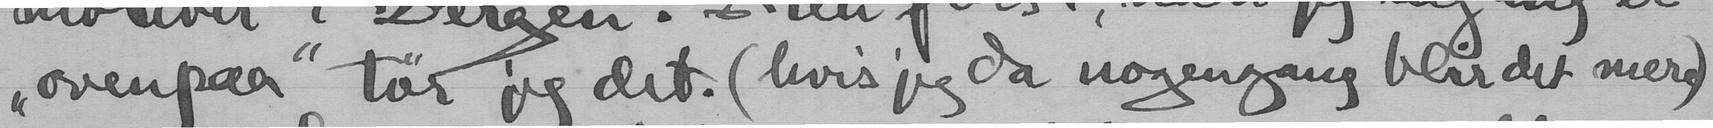"ovenpaa" tør jeg det, (hvis jeg da nogengang blir det mere)
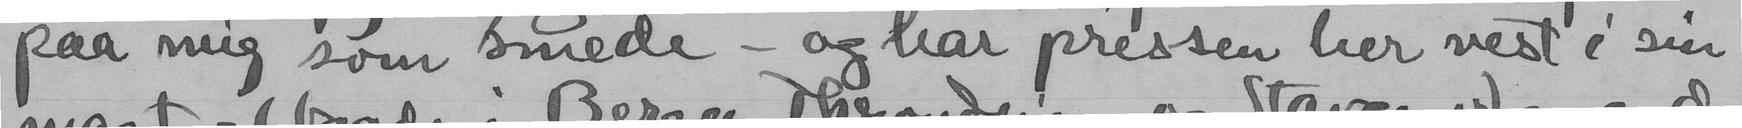paa mig som smede - og har pressen her vest i sin
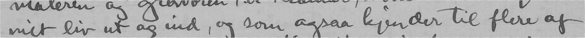mit liv ut og ind, og som ogsaa kjender til flere af
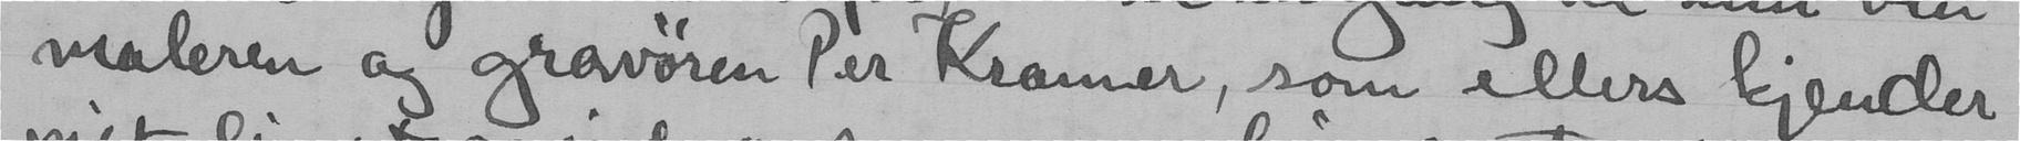maleren og gravøren Per Kramer, som ellers kjender
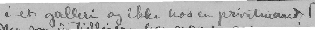i et gallere og ikke hos en privatmand
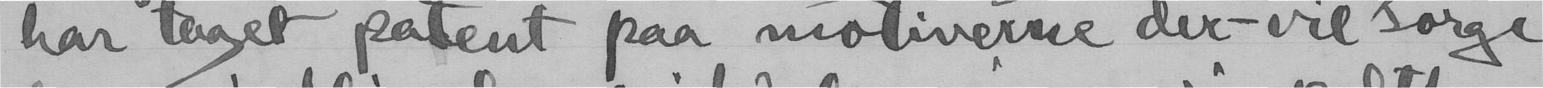har taget patent paa motiverne der - vil sørge
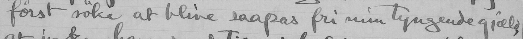først søke at blive saapas fri min tyngende gjæld,
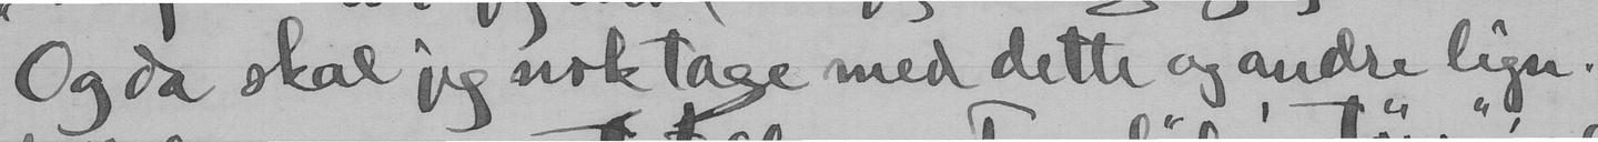Og da skal jeg nok tage med dette og andre lign.
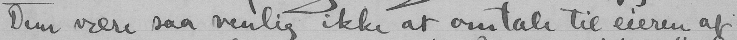Dem være saa venlig ikke at omtale til eieren af
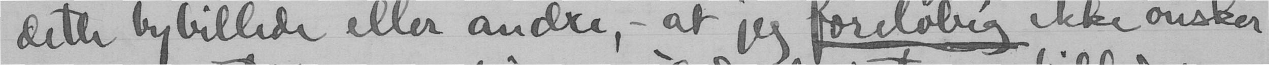dette bybillede eller andre - at jeg foreløbig ikke ønsker
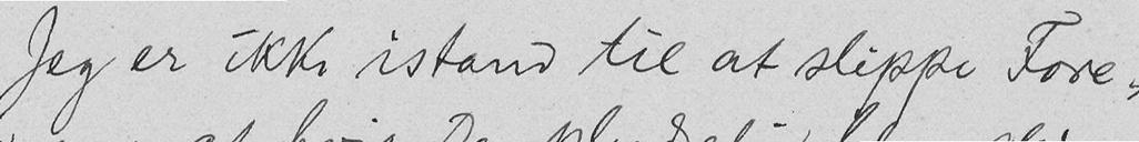Jeg er ikke istand til at slippe Fore-
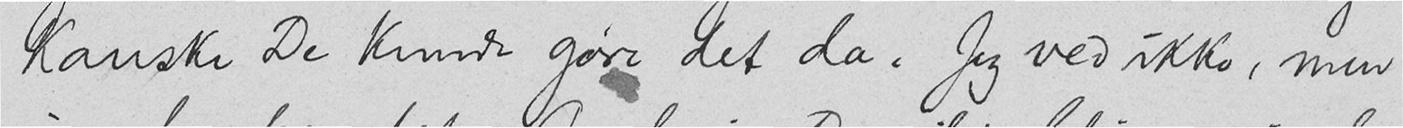kanske De kunde gøre det da. Jeg ved ikke, men
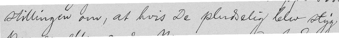stillingen om, at hvis De pludselig blev styg
